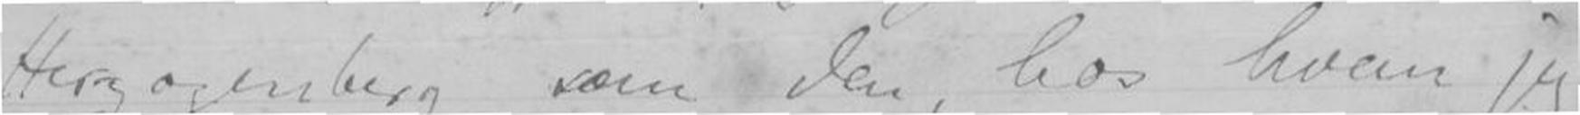Herzogenberg som den, hos hvem jeg
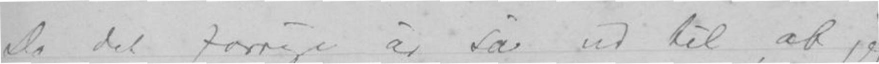Da det forige år så ud til at jeg
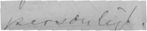personligt.
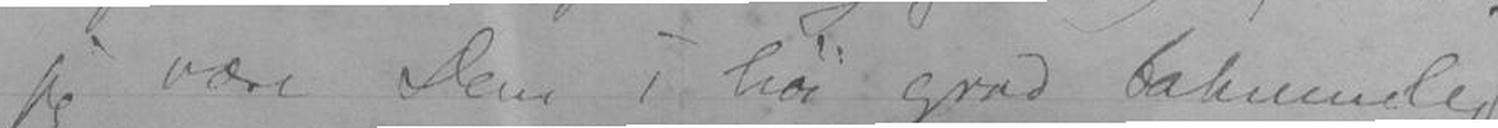jeg være Dem i høi grad taknemlig
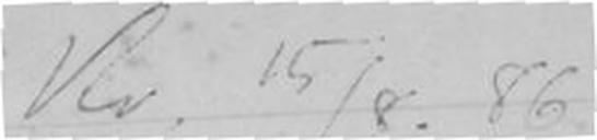Kr. 15/8.86.
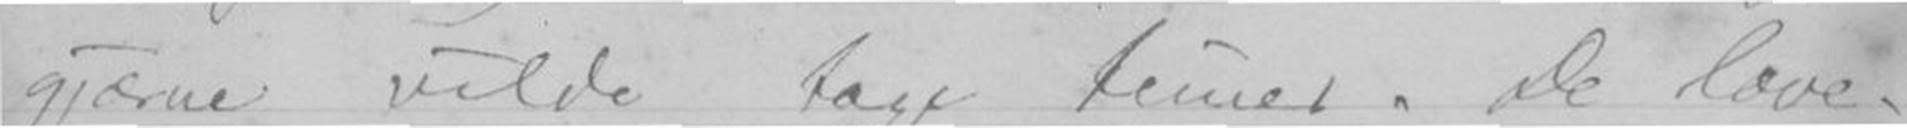gjærne vilde tage timer. De love-
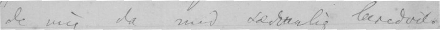de mig da med sædvanlig beredvil-
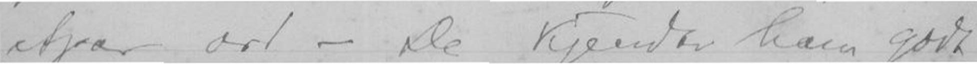etpar ord - De kjendte ham godt
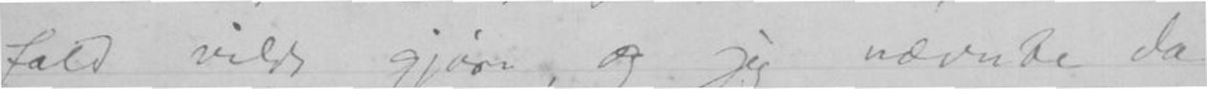fald vilde gjøre, og jeg nævnte da
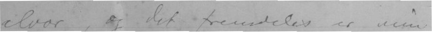alvor, og det fremdeles er min
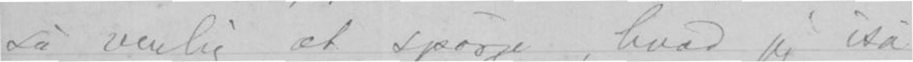så venlig at spørge, hvad jeg iså-
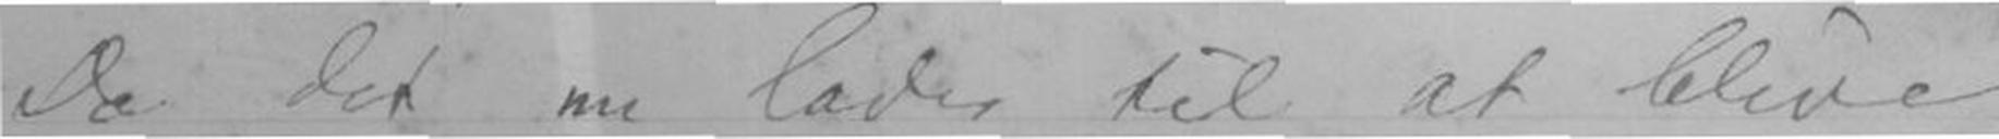Da det nu lader til at blive
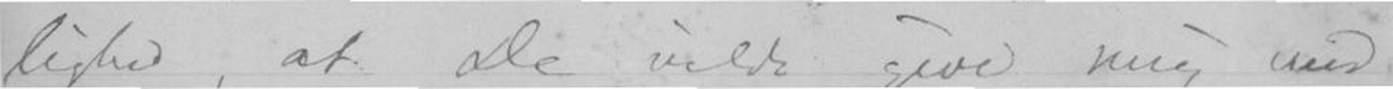lighed, at De vilde give mig med
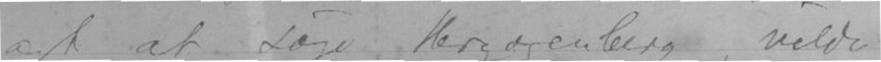agt at søge Herzogenberg, vilde
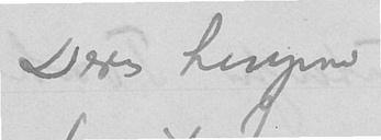Deres hengivne
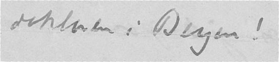doktoren i Bergen!
